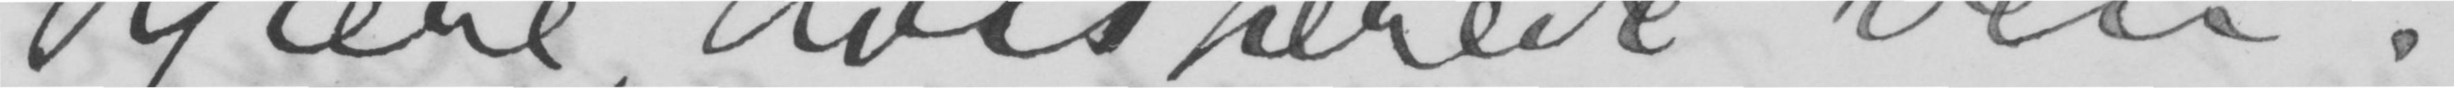Kjære høistærede ven!
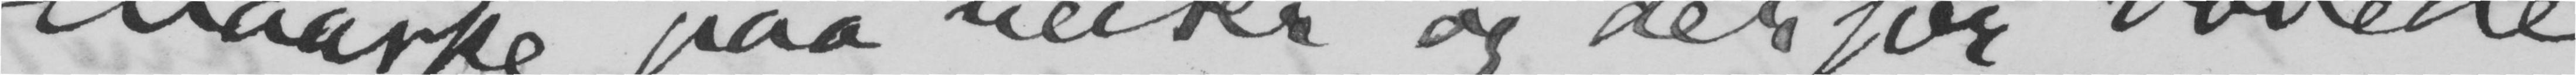maaske paa reiser, og derfor vovede
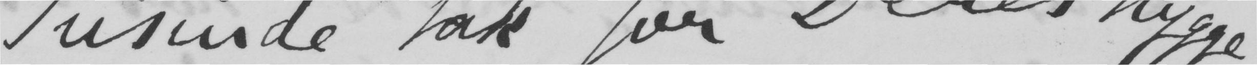Tusinde tak for Deres hygge-
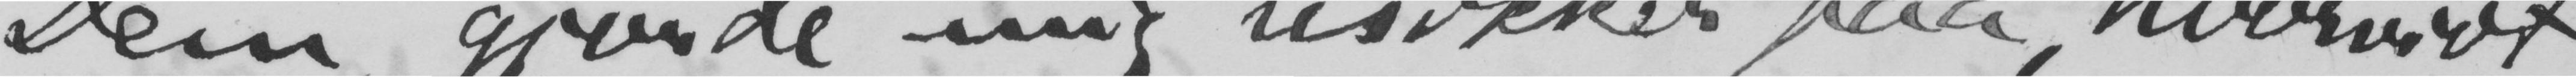Dem, gjorde mig usikker paa, hvorvidt
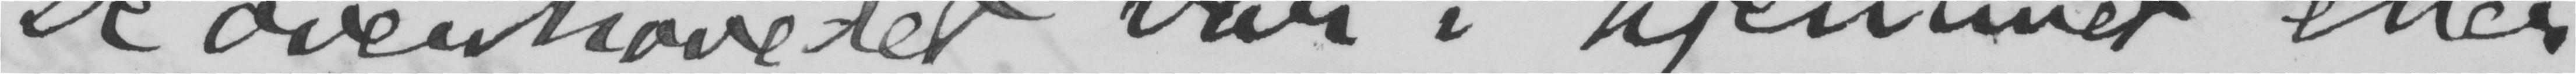De overhovedet var i hjemmet eller
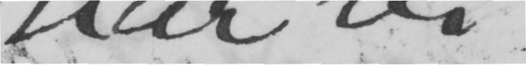har vi
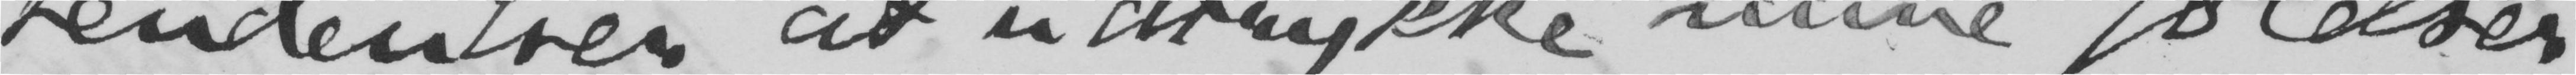tendentser at udtrykke mine følelser
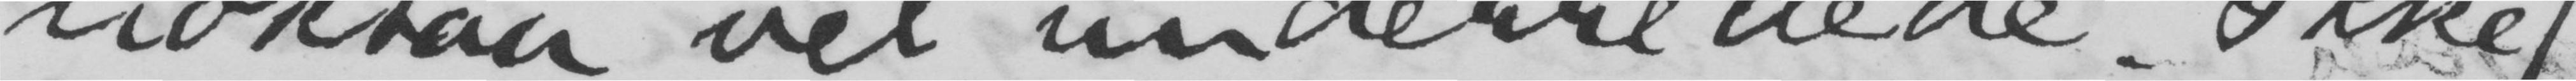noksaa vel underrettede. Ikke
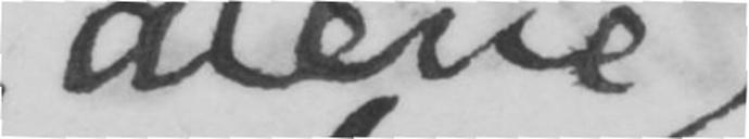alene
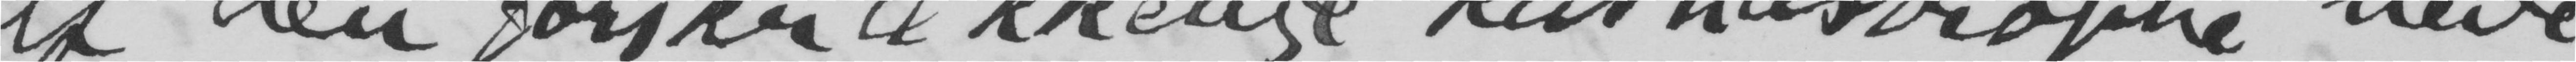af den forskrækkelige katastrophe nede
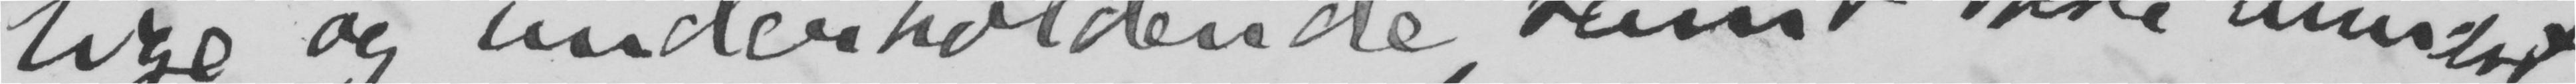lige og underholdende, samt ikke mindst
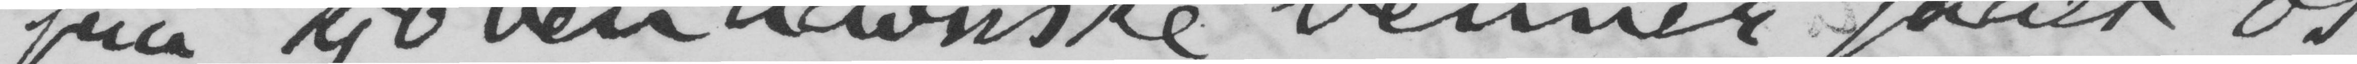fra kjøbenhavnske venner faaet os
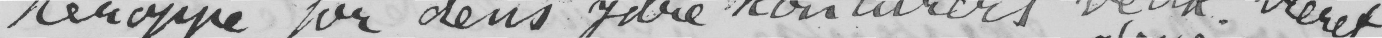heroppe for dens ydre konturers vedk. været
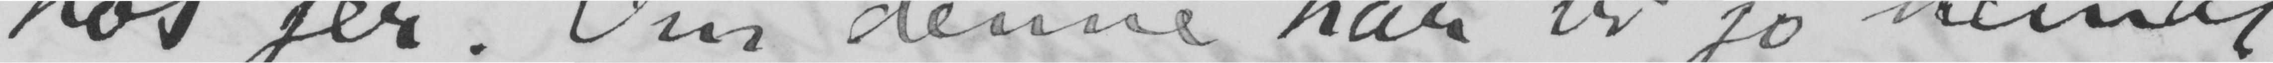hos jer. Om denne har vi jo nemlig
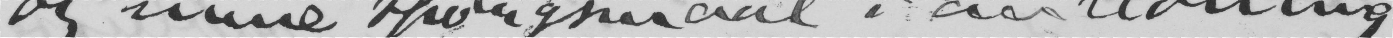og mine spørgsmaal i anledning
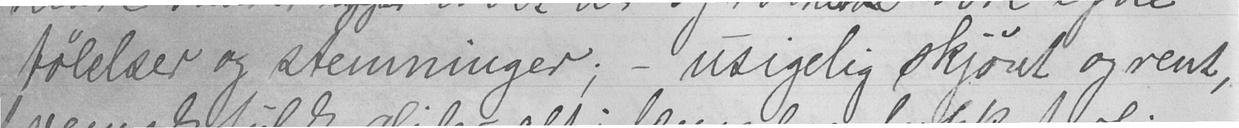følelser og stemninger; - usigelig skjønt og rent,
